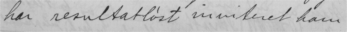har resultatløst inviteret ham
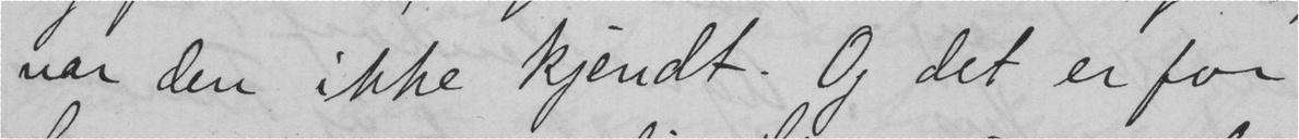var den ikke kjendt. Og det er for
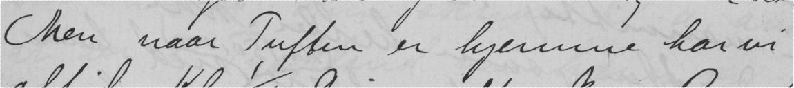Men naar Tuften er hjemme har vi
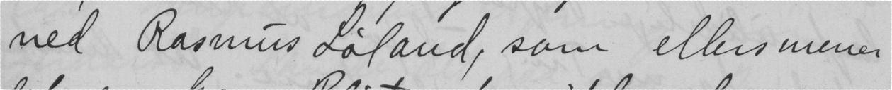ned Rasmus Løland, som ellers mener
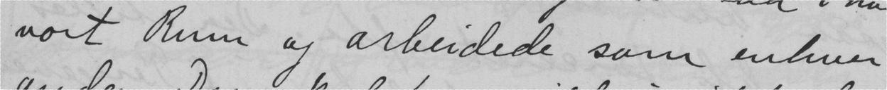vort Rum og arbeidede som enhver
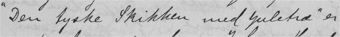"Den tyske Skikken med Juletræ" er
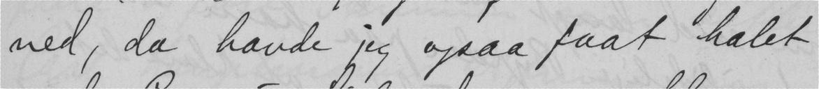ned, da havde jeg ogsaa faat halet
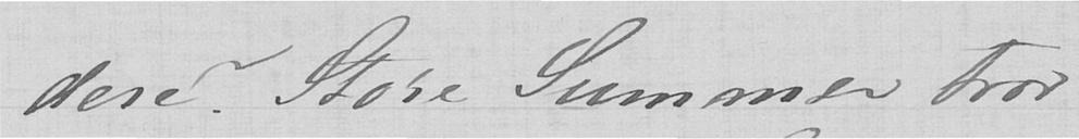dere? Store Summer tror
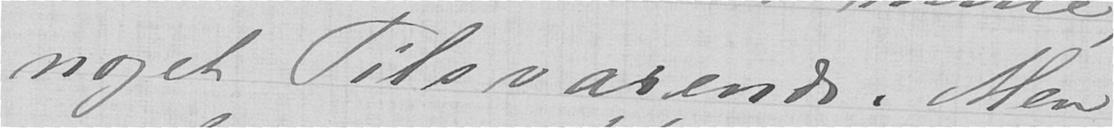noget Tilsvarende. Men
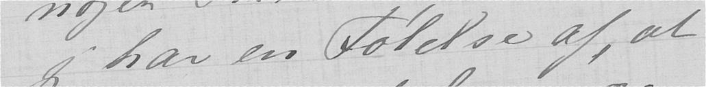jeg har en Følelse af, at
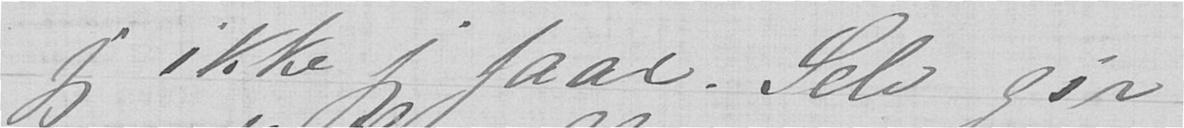jeg ikke jeg faar. Selv gir
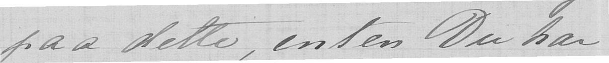paa dette, enten Du har
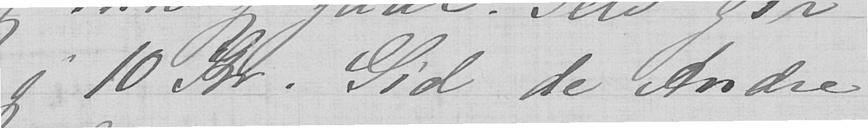jeg 10 Kr. Gid de Andre
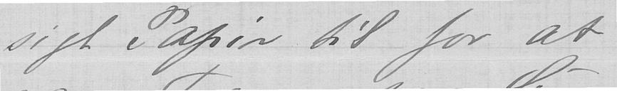sigt Papir til for at
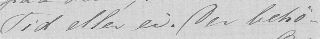Tid eller er. Der behø-
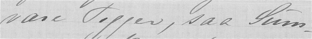være Tigger, saa Sum-
